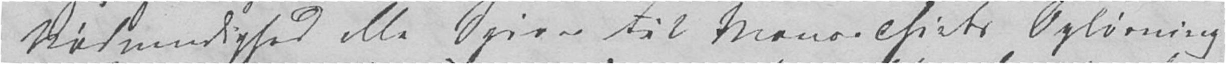Nødvendighed alle Spirer til Monarchiets Opløsning
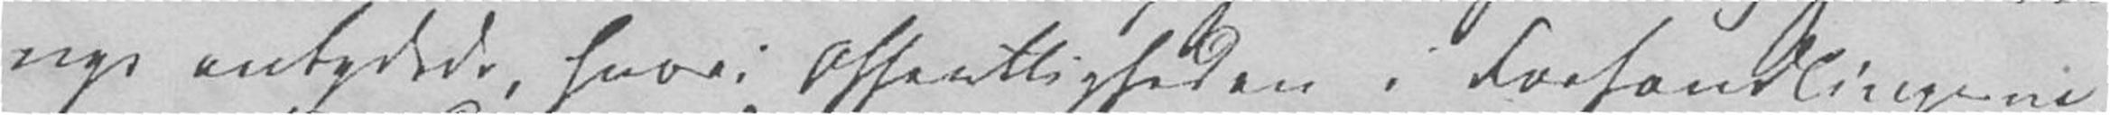nys antydede, hvori Offentligheden i Forhandlingerne,
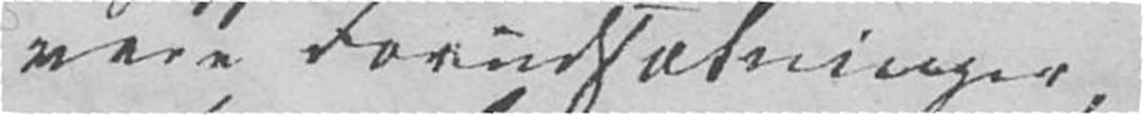vare Forudsætninger,
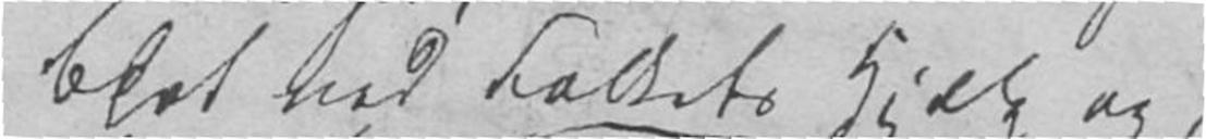blot ved Folkets Hjælp og
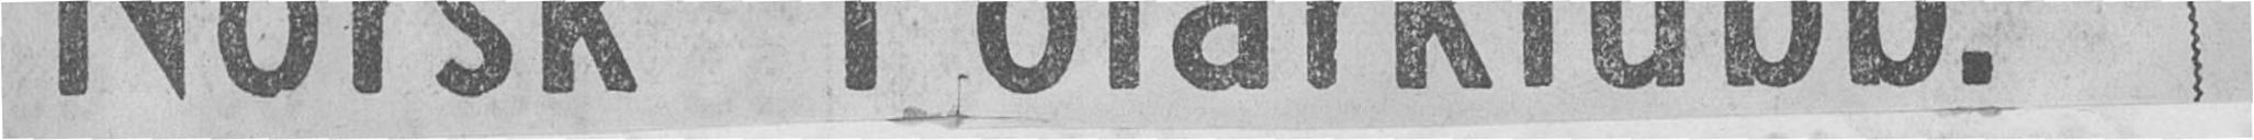Norsk Polarklubb.
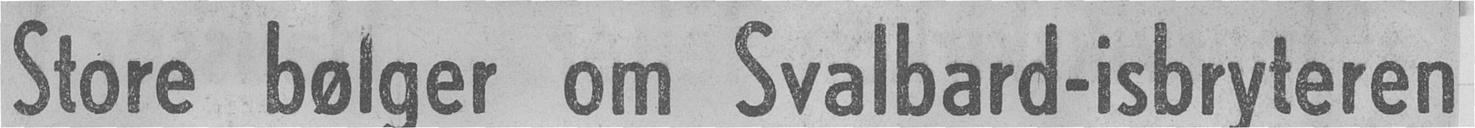Store bølger om Svalbard-isbryteren
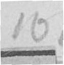167
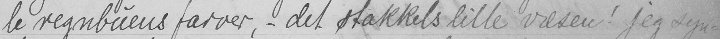le regnbuens farver, - det stakkels lille væsen! jeg syn-
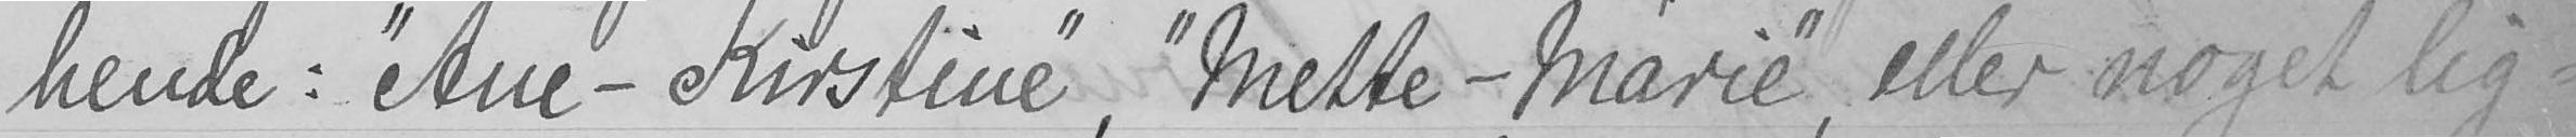hende: "Ane-Kirstine", Mette-Marie", eller noget lig-
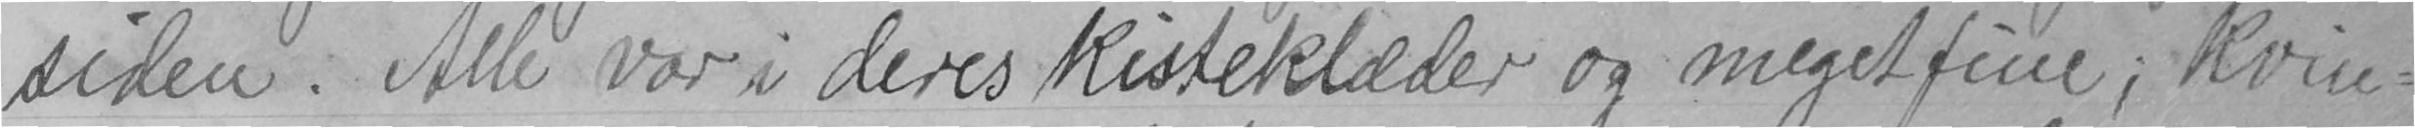siden: Alle vor i deres kisteklæder og meget fine; kvin-
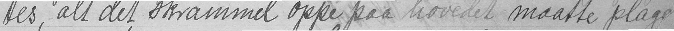tes, alt det skrammel oppe paa hovedet maatte plage
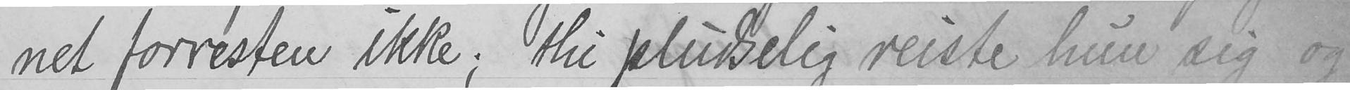net forresten ikke; thi pludselig reiste hun sig og
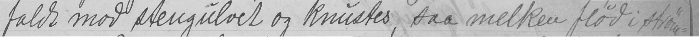faldt mod stengulvet og knustes, saa melken flød i strøm-
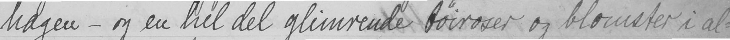hagen - og en hel del glimrende tøiroser og blomster i al-
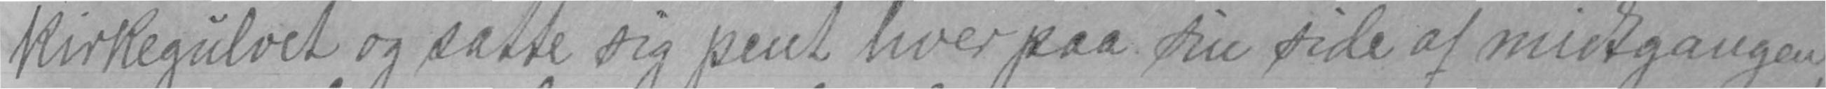kirkegulvet og satte sig pent hver paa sin side af midtgangen,
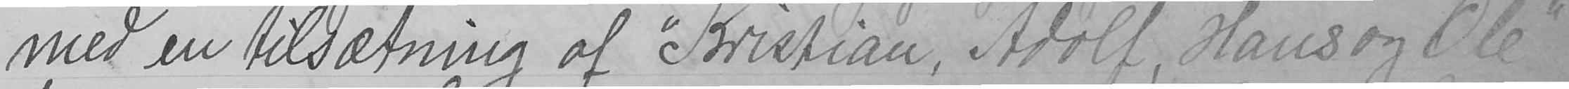med en tilsætning af "Kristian, Adolf, Hans og Ole"
